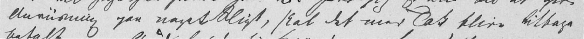Anviisning paa noget Sligt, skal det med Tak blive tilbage
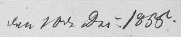Den 10de Dec. 1855
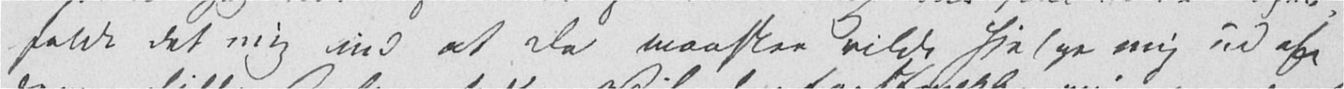faldt det mig ind at De maaaske vilde hjelpe mig ud af
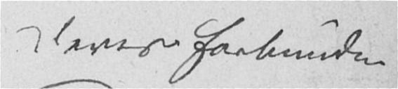Deres forbundne
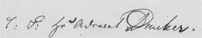S: T: Hr Advocat Dunker.
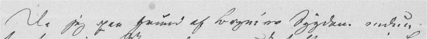Da jeg paa Grund af Brynies Sygdom endnu
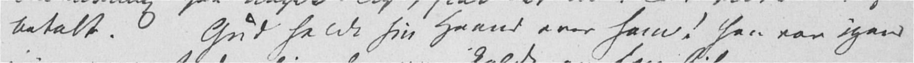betalt. Gud holde sin Haand over ham! Han var igaar
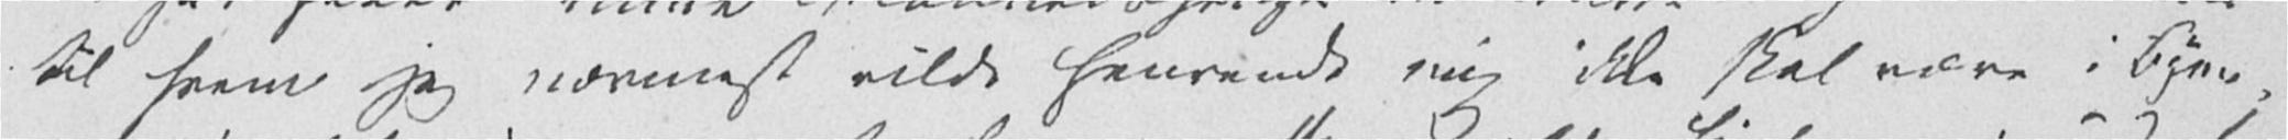til hvem jeg nærmest vilde henvende mig ikke skal være i Byen,
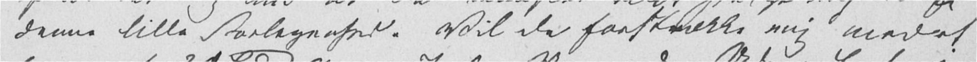denne lille Forlegenhed. Vil De forstrække mig med et
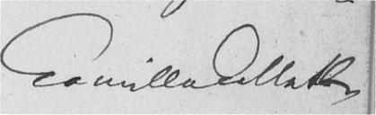Camilla Collett
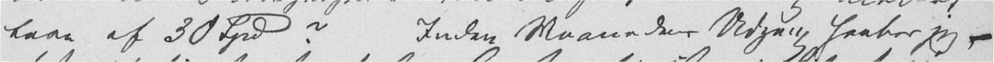Laan af 30 Spd? Inden Maanedens Udgang haaber jeg –
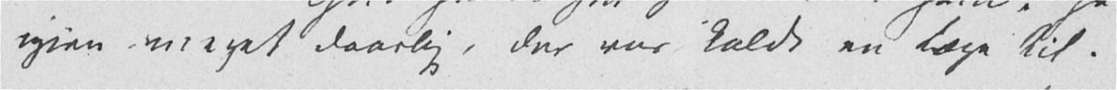igjen meget daarlig, der var kaldt en Læge til.
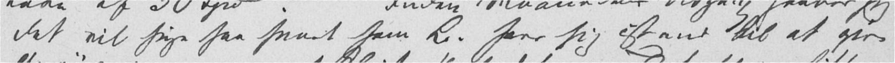det vil sige saa snart som B. seer sig istand til at give
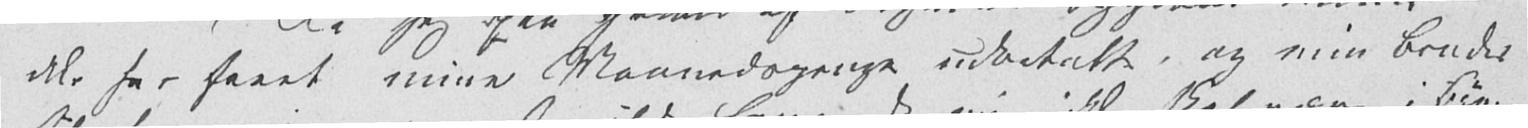ikke har faaet mine Maanedspenge udbetalte, og min Broder
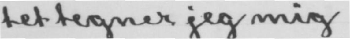tet tegner jeg mig
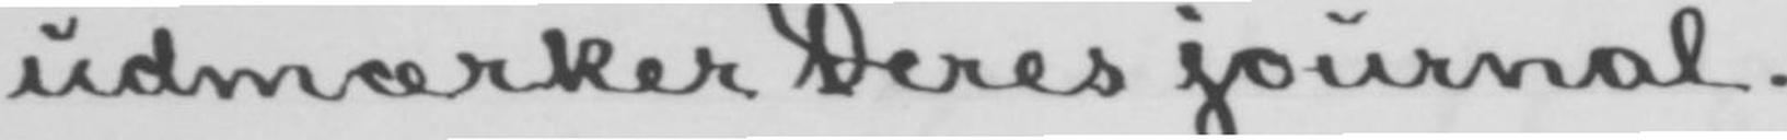udmærker Deres journal.
